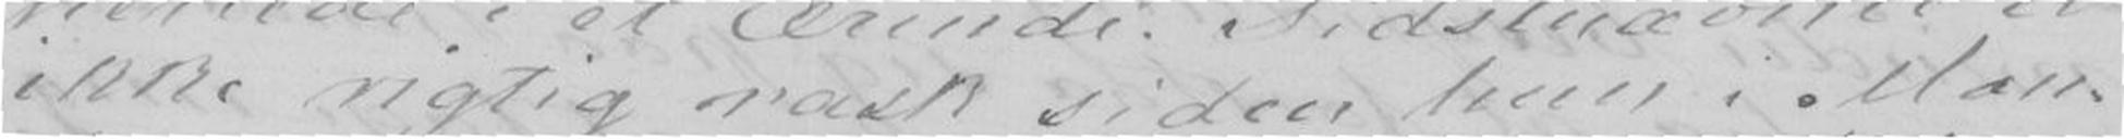ikke rigtig rask siden hun i Man-
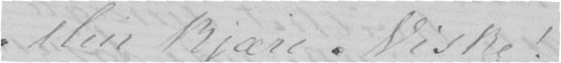Min kjære Niske!
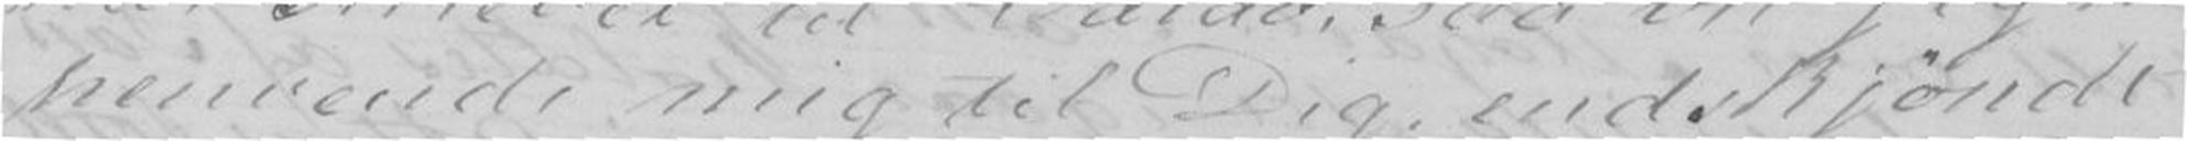henvende mig til Dig, endskjøndt
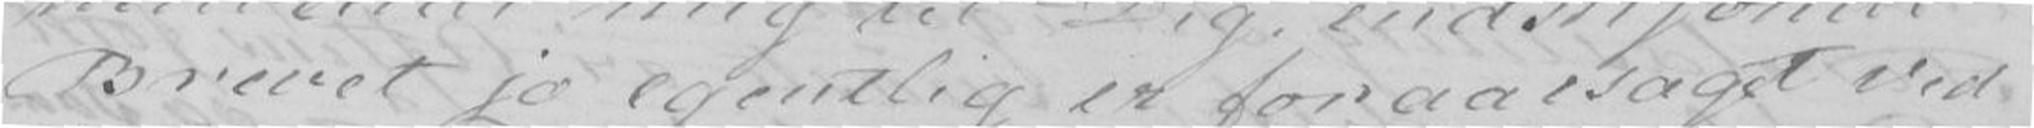Brevet jo egentlig er foraarsaget ved
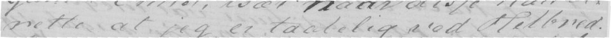rette at jeg er taalelig ved Helbred.
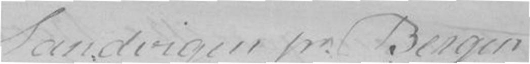Sandvigen pr. Bergen
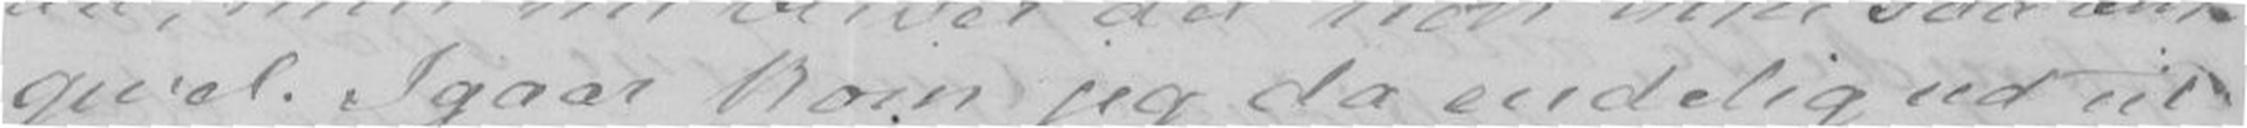gevel. Igaar kom jeg da endelig ud til
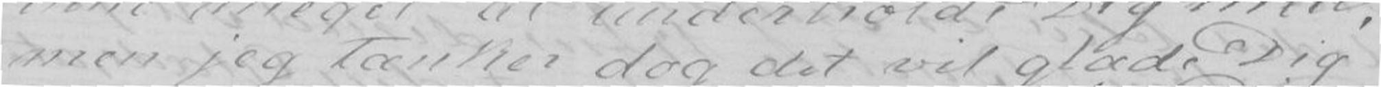men jeg tænker dog det vil glæde Dig
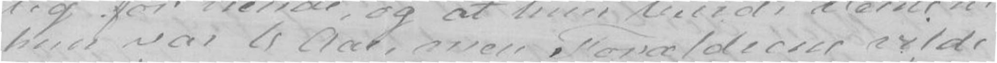hun var 6 Aar, men Forældrene vilde
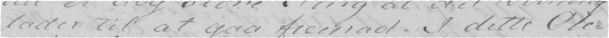lader til at gaa fremad. I dette Øie-
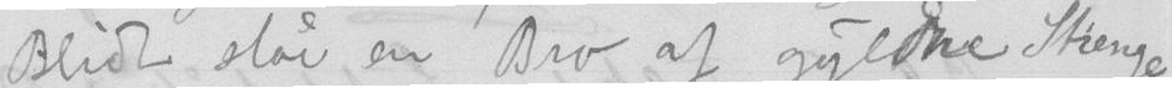Blidt slår en Bro af Gylne Strenge
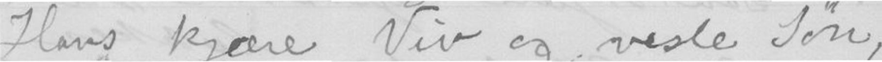Hans kjære Viv og vesle Søn,
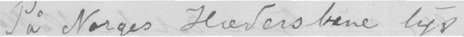På Norges Hædersbane lys,
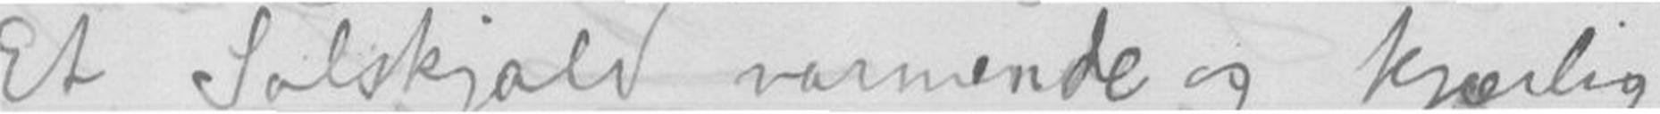Et Solskjold varmende og kjærlig
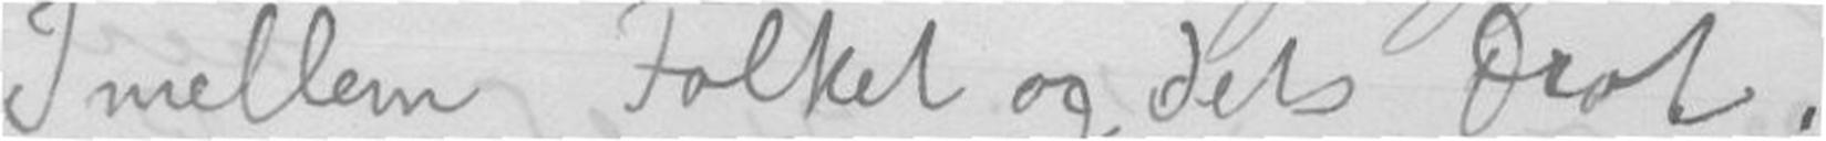Imellem Folket og dets Drot.
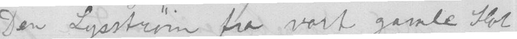Den Lysstrøm fra vort gamle Slot
